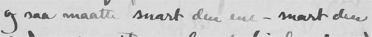og saa maatte snart den ene - snart den
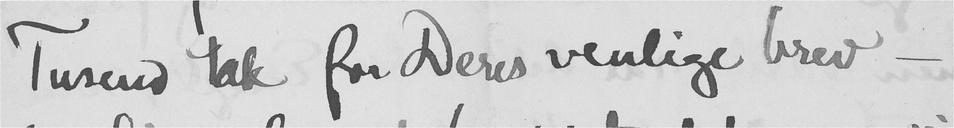Tusend tak for Deres venlige brev -
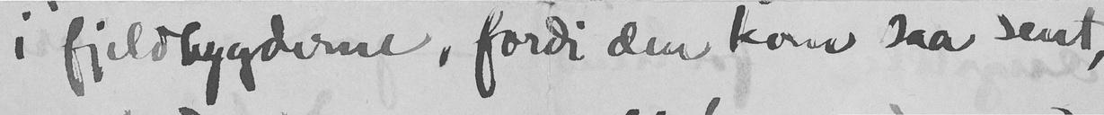i fjeldbygderne, fordi den koms saa sent,
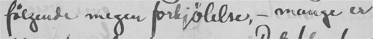følgende megen forkjølelse, - mange er
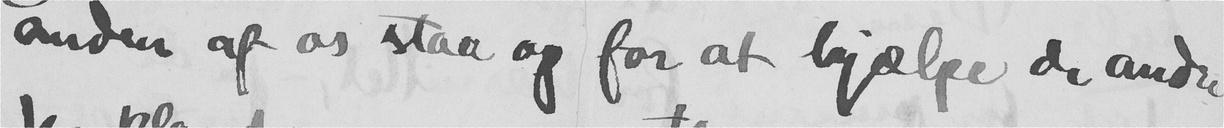anden af os staa op for at hjælpe de andre
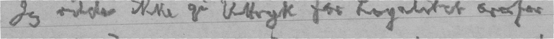Jeg vilde ikke gi Uttryk for Loyalitet overfor
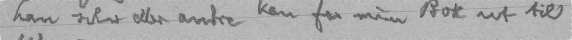han selv eller andre kan faa min Bok ut til
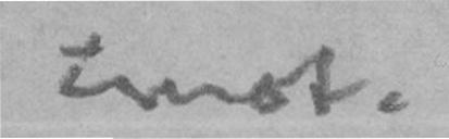imot.
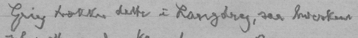Grieg trækker dette i Langdrag, saa hverken
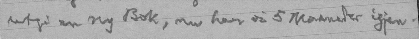utgi en ny Bok, nu har vi 5 Maaneder igjen.
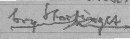bry Stortinget.
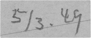5/3. 49
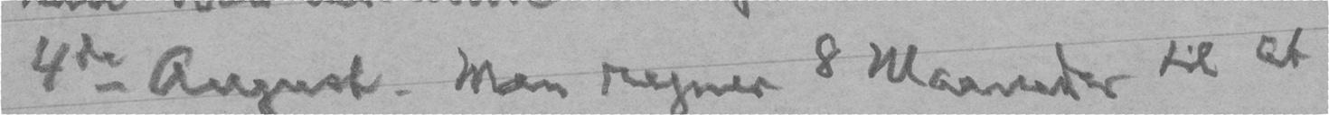4de August. Man regner 8 Maaneder til at
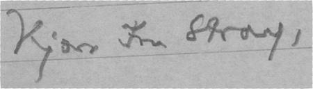Kjære Fru Stray,
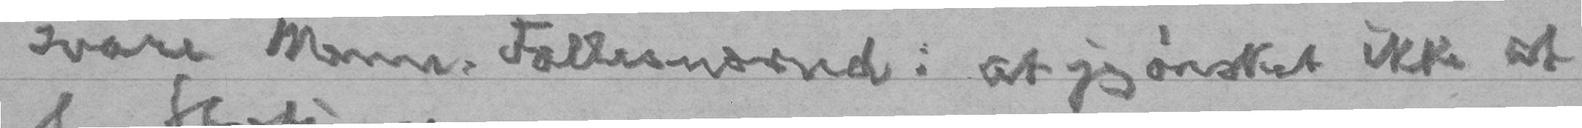svare Menn. Fællesnævnd: at jeg ønsket ikke at
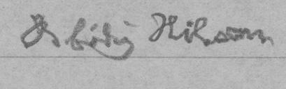Ærbødig Hilsen
